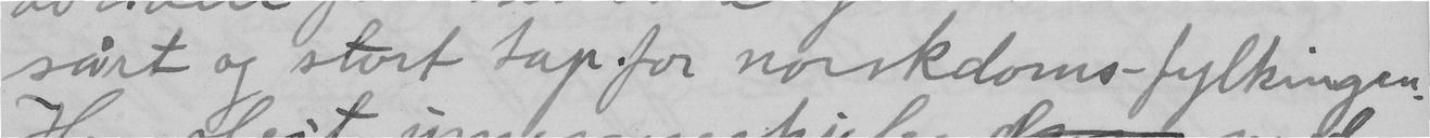sårt og stort tap for norskdoms-fylkingen.
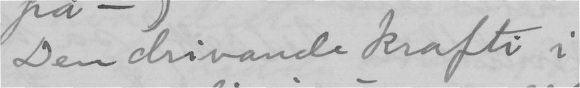Den drivande krafti i
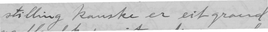stilling kanske er eit grand
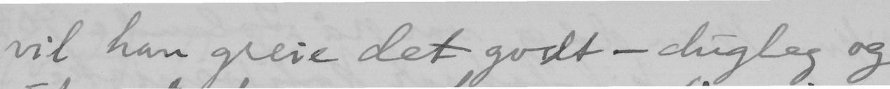vil han greie det godt – dugleg og
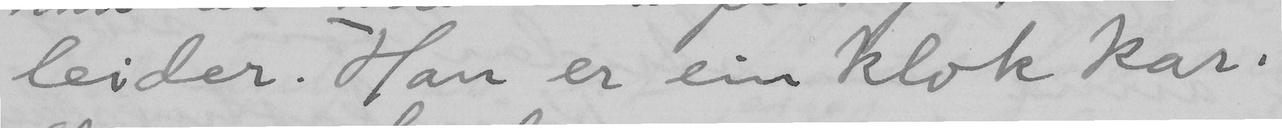leider. Han er ein klok kar.
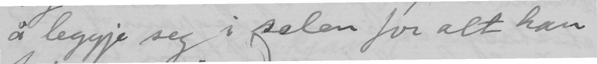å leggje seg i selen for alt han
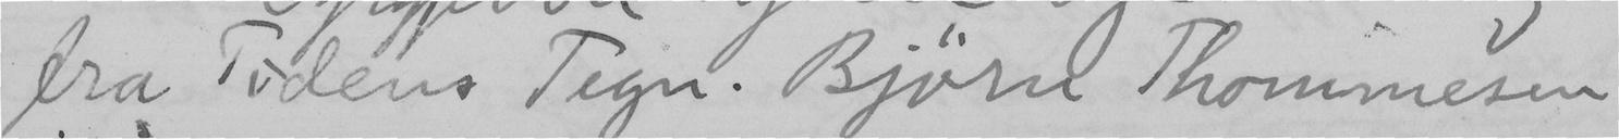fra Tidens Tegn. Björn Thommesen
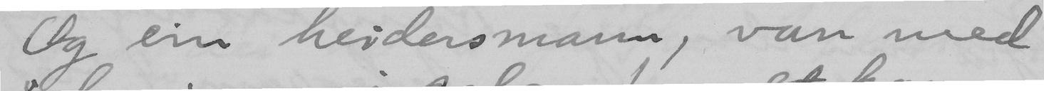Og ein heidersmann, van med
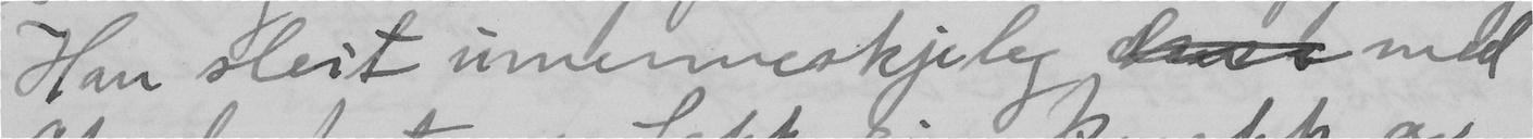Han sleit umenneskjeleg med
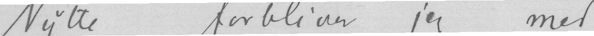Nytte forbliver jeg med
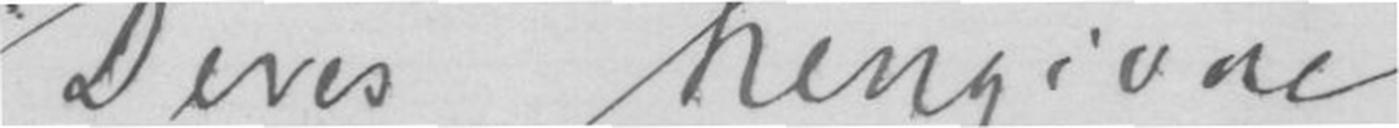Deres hengivne
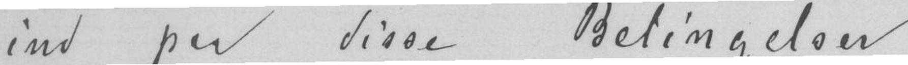ind paa disse Betingelser.
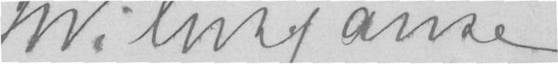Wilhelm Hansen
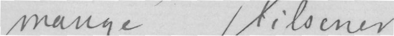mange hilsener
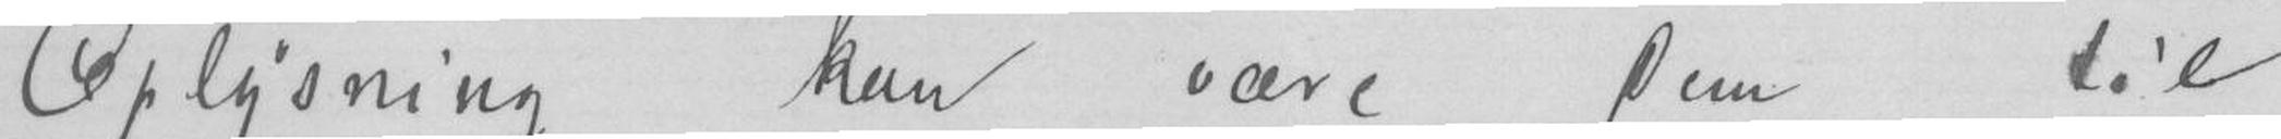Oplysning kan være Dem til
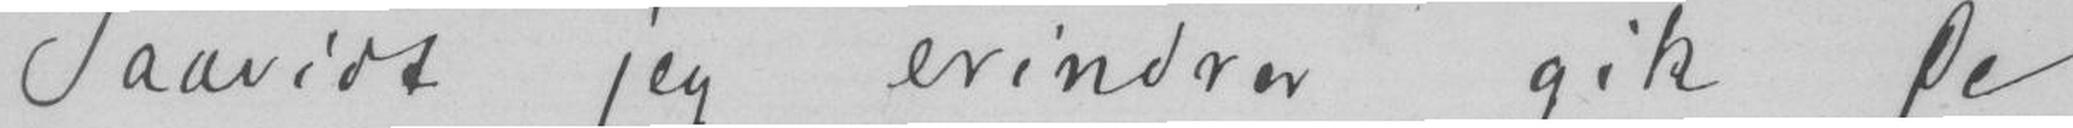Saavidt jeg erindrer gik de
